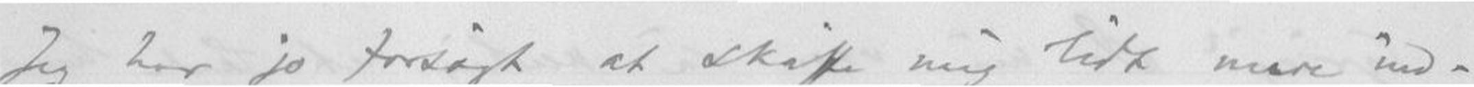Jeg har jo forsøgt at skaffe mig lidt mere ind-
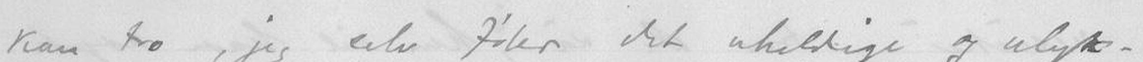kan tro, jeg selv føler det uheldige og ulyk-
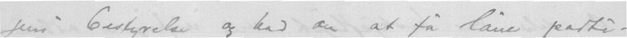gens" bestyrelse og bad om at få låne parti-
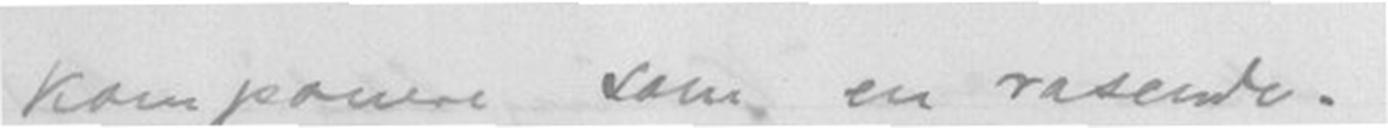komponere som en rasende.
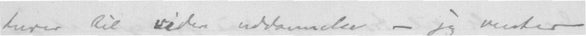turer til videre uddannelser - jeg venter
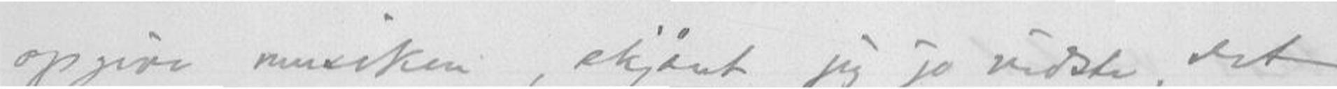opgive musiken, skjønt jeg jo vidste, det
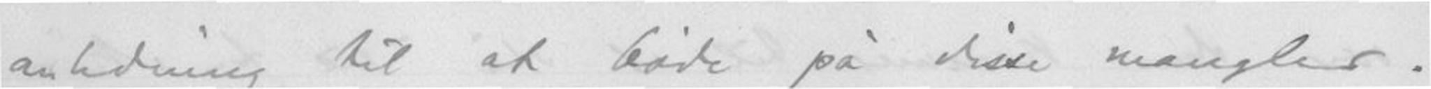anledning til at bøde på disse mangler.
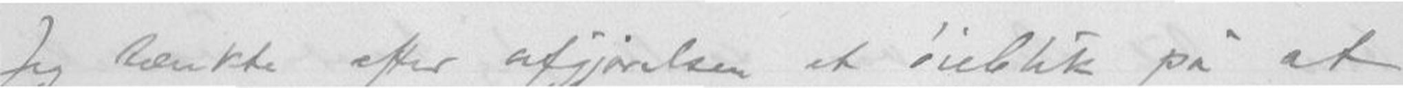Jeg tænkte efter afgjørelsen et øieblik på at
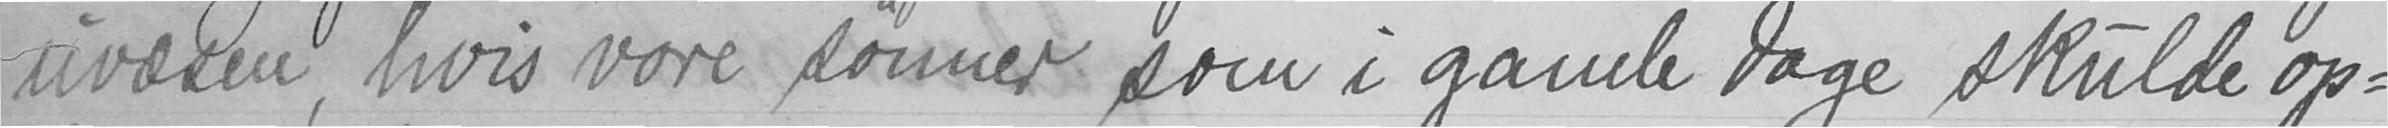uvæsen, hvis vore sønner som i gamle dage skulde op=
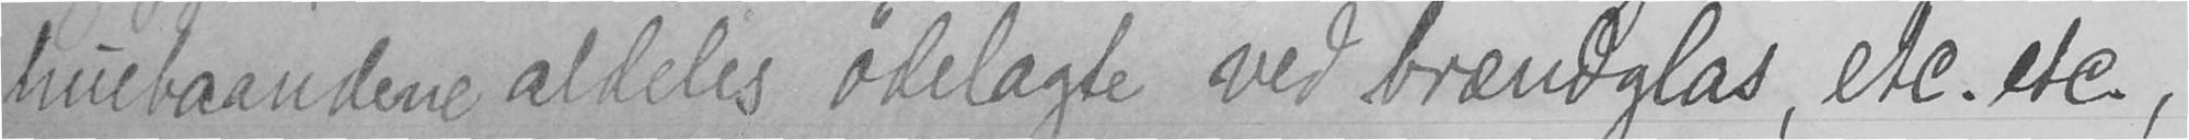huebaandene aldeles ødelagte ved brændglas, etc. etc.,
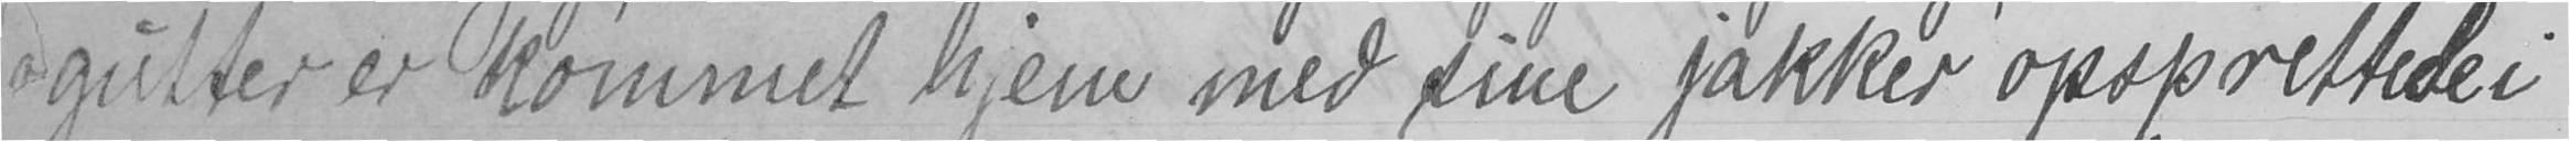gutter er kommet hjem med sine jakker opsporettede i
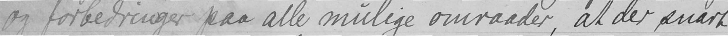og forbedringer paa alle mulige omraader, at der snart
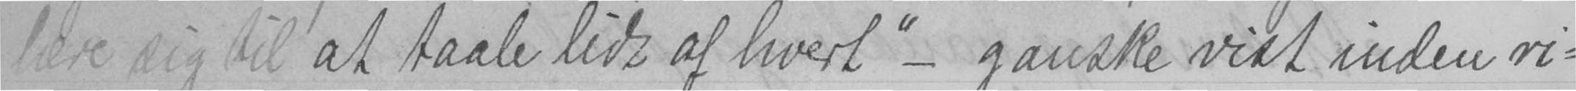lære sig til at taale lidt af hvert" - ganske vist inden ri-
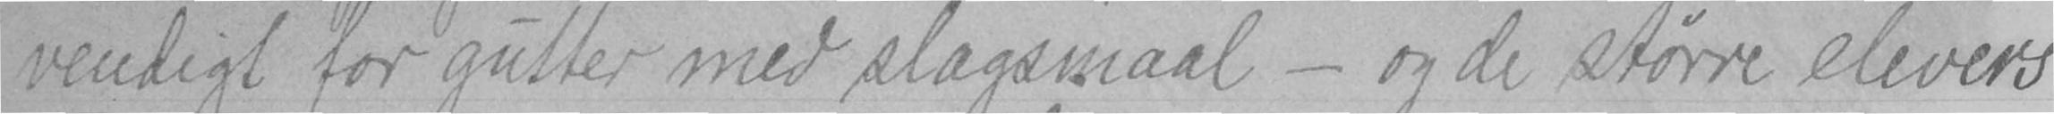vendigt for gutter med slagsmaal - og de større elevers
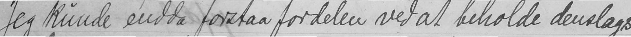Jeg kunde endda forstaa fordelen ved at beholde denslags
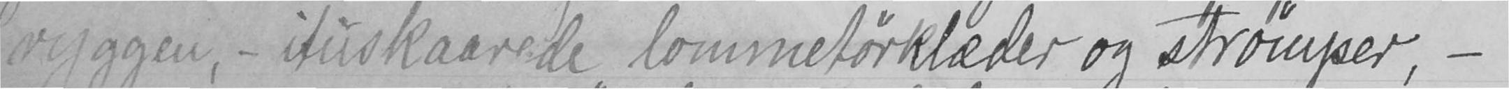ryggen, - ituskaarede lommetørklæder og strømper, -
